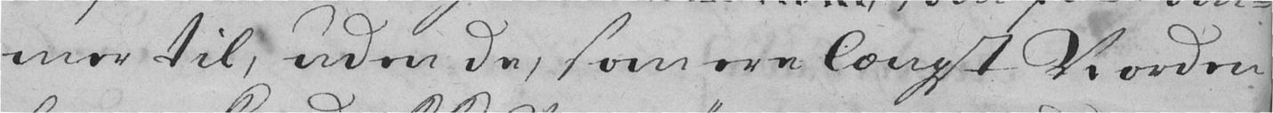mer til, uden de, som ere længst Norden
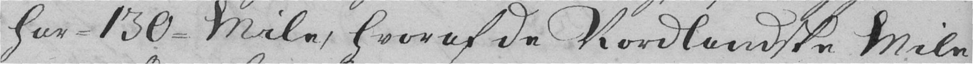har 130 Mile, hvoraf de Nordlandske Mile
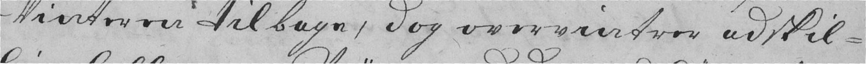Vinteren tilbage, dog overvintrer adskil-
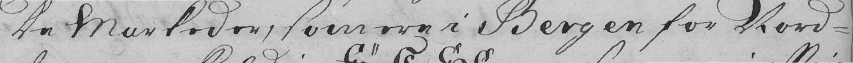De Markeder, som ere i Bergen for Nord-
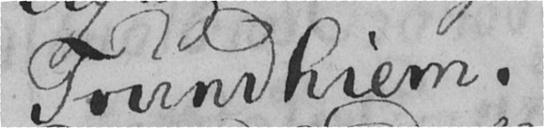Trundhiem.
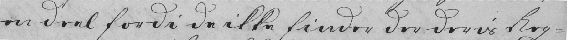en deel fordi de ikke finder der deris Reg-
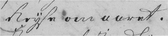Reyse om aaret.
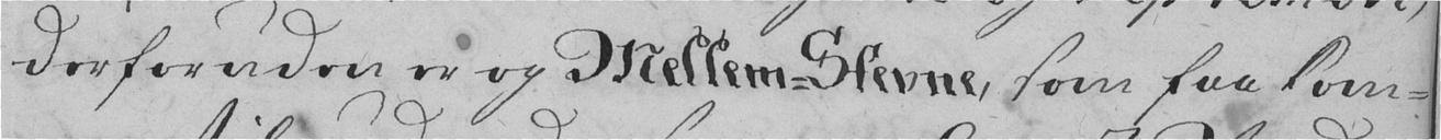derforuden er og Mellem-Stevne, som faa Som-
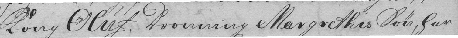Kong Oluf, Dronning Margrethes Søn, har
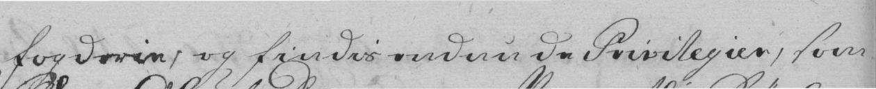Fogderie, og findis endnu de Privilegier, som
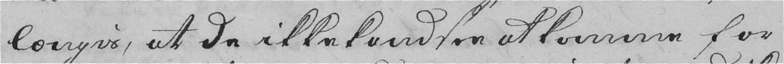længis, at de ikke kand see at komme for
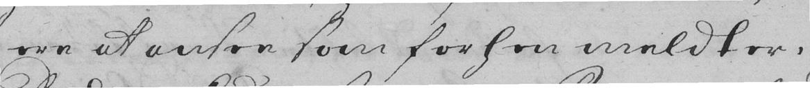ere at ansee som for hen meldter.
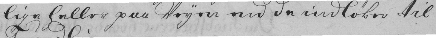lige heller paa Veyen end de indløber til
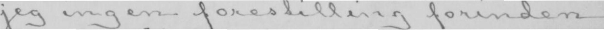jeg ingen forestilling forinden
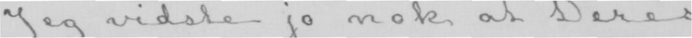Jeg vidste jo nok at Deres
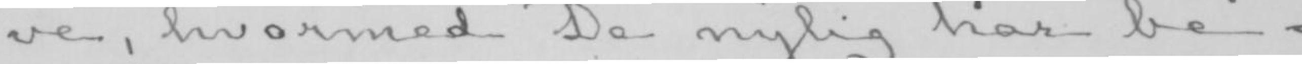ve, hvormed De nylig har be-
